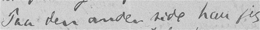Paa den anden side har jeg
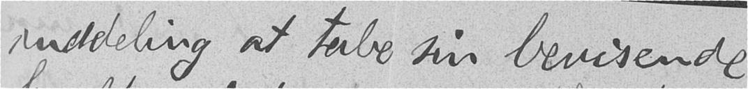inddeling at tabe sin bevisende
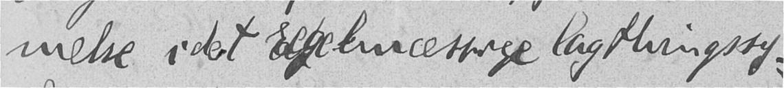melse i det regelmæssige lagthingssy-
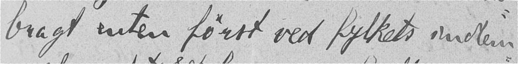bragt enten først ved fylkets indlem-
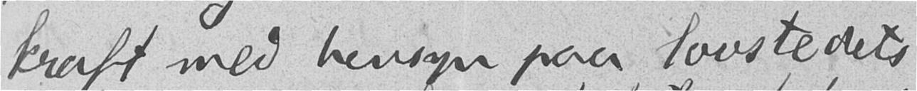kraft med hensyn paa lovstedets
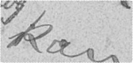kan
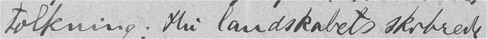tolkning; thi landskabets skibrede-
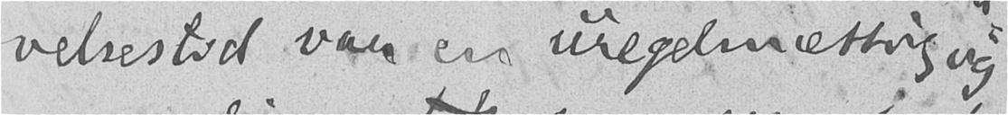velsestid var en uregelmæssig og
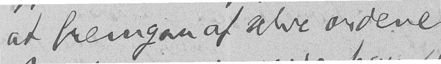at fremgaa af selve ordene.
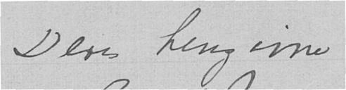Deres hengivne
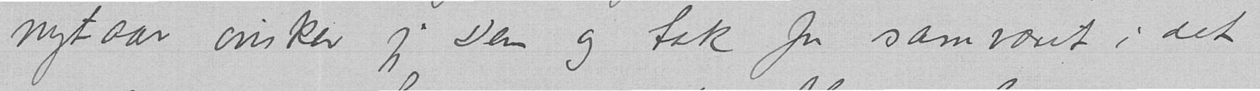nytaar ønsker jeg Dem og tak for samværet i det
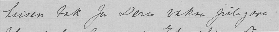tusen tak for Deres vakre julegave!
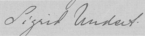Sigrid Undset.
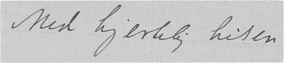Med hjertelig hilsen
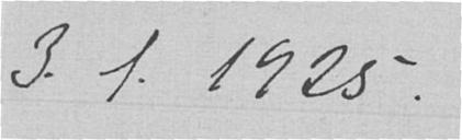3.1.1925.
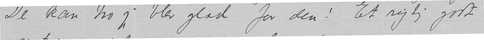De kan tro jeg blev glad for den! Et rigtig godt
